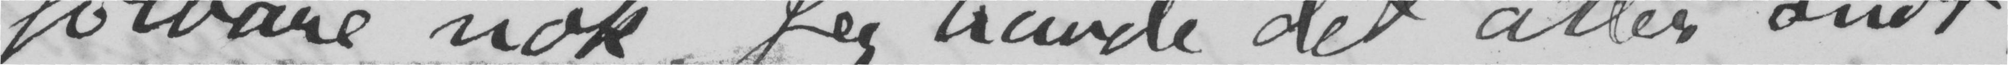følbare nok. Jeg havde det atter ondt.
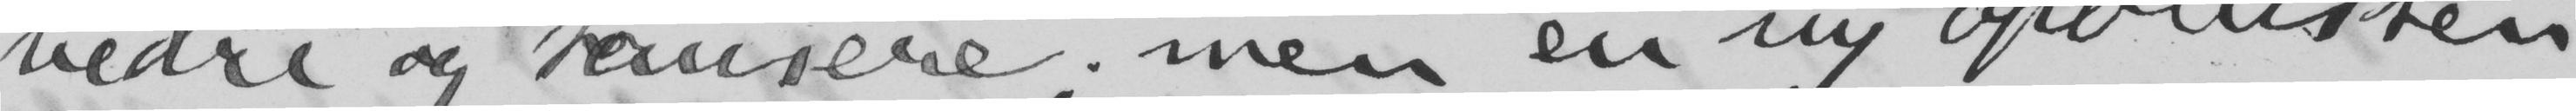bedre og tausere; men en ny opblussen
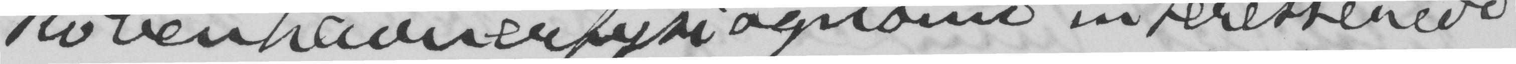Københavnerfysiognomi interesserede
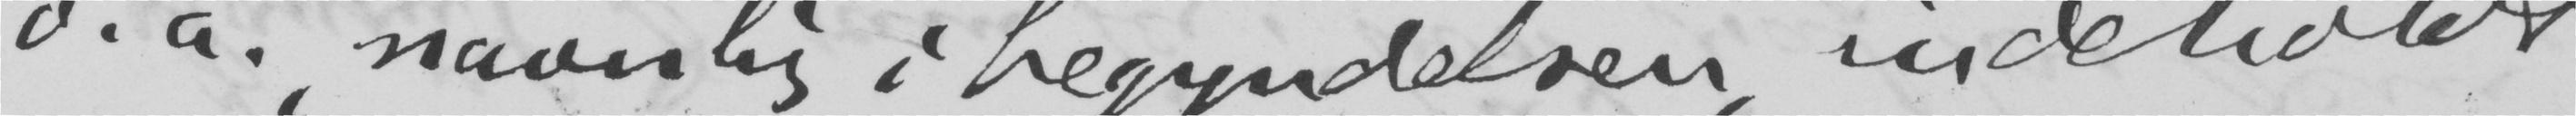o.a. navnlig i begyndelsen, indeholdt
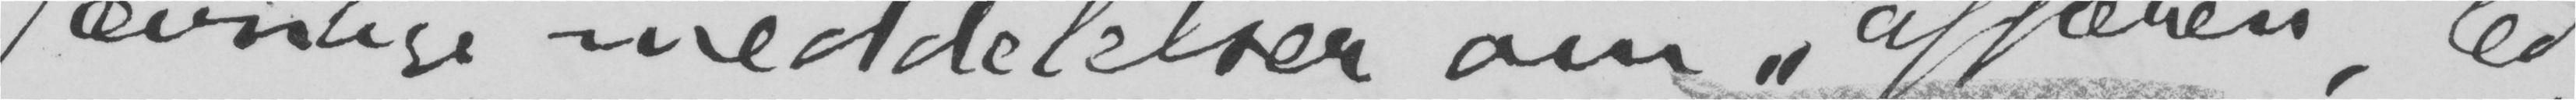jævnlige meddelelser om «affæren», led-
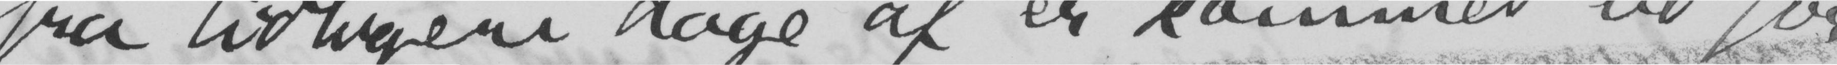fra tidligere dage af er kommet ud for
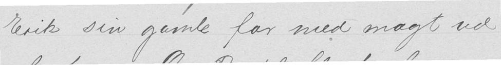Erik sin gamle far med magt ud
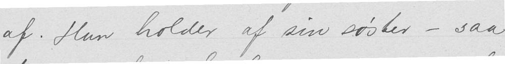af. Han holder af sin søster - saa
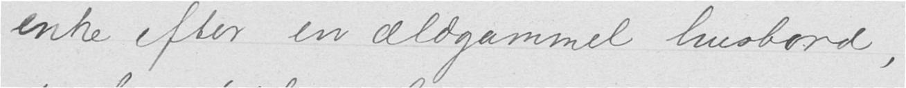enke efter en ældgammel husbond,
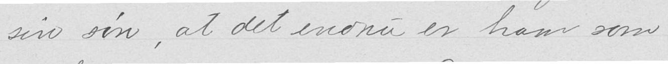sin søn, at det endnu er ham som
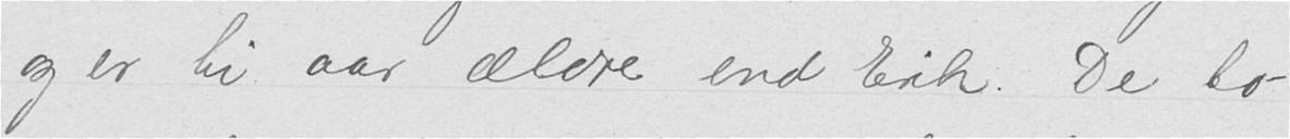og er ti aar ældre end Erik. De to
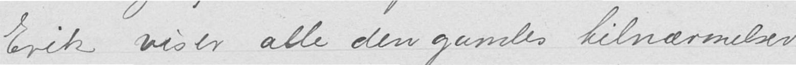Erik viser alle den gamles tilnærmelser
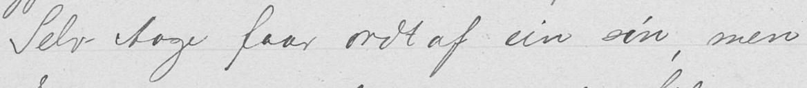Selv Aage faar ondt af sin søn, men
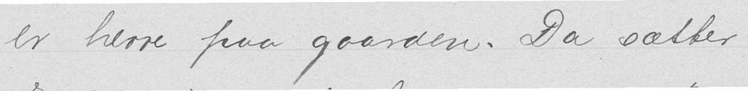er herre paa gaarden. Da sætter
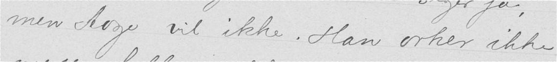men Aage vil ikke. Han orker ikke
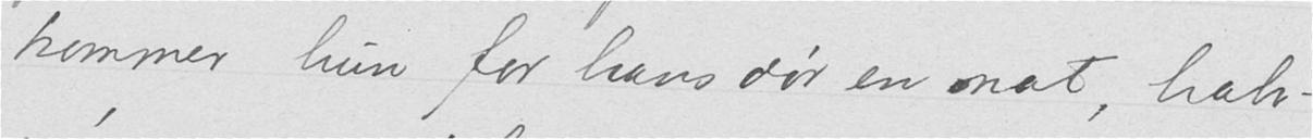kommer hun for hans dør en nat, halv-
